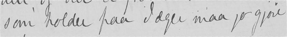som holder paa Jæger maa jo gjøre
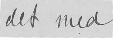det med
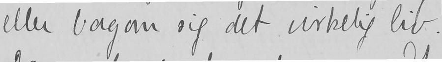eller bagom sig det virkelig liv.
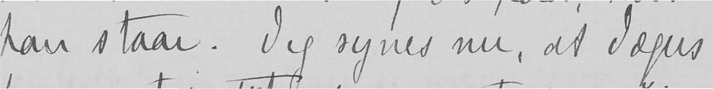han staar. Jeg synes nu, at Jægers
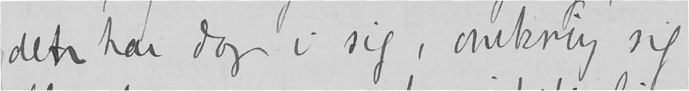den har dog i sig, omkring sig
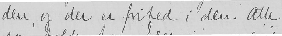den, og der er frihed i den. Alle
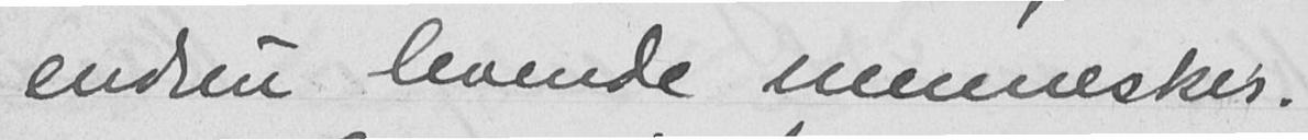endnu levende mennesker.
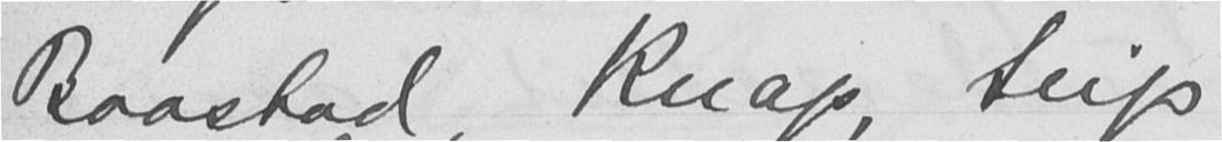Baastad, Knap, Seip
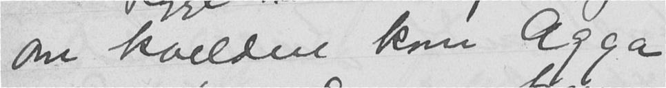Om kvelden kom Agga
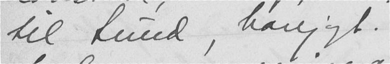til Sund, harejagt.
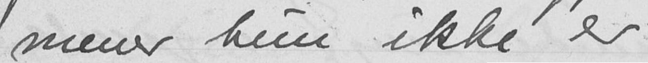mener hun ikke er
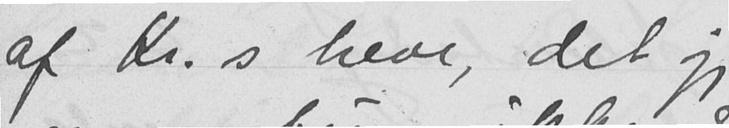af Kr. s breve, det jeg
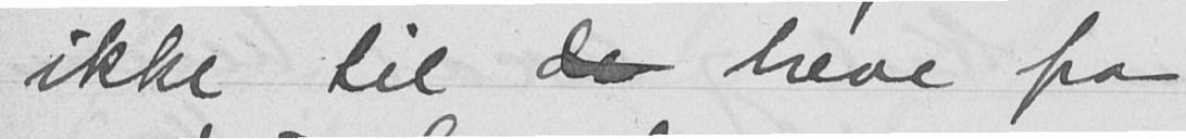ikke til de breve fra
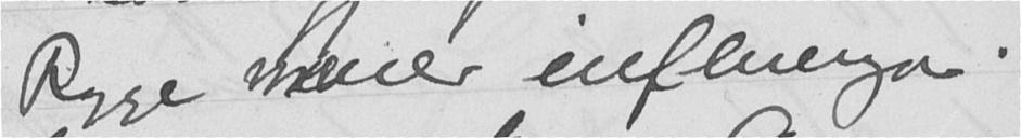Rogge mener influenza.
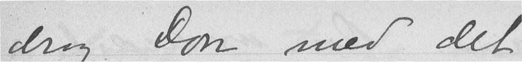drog Don med det
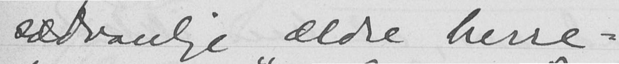sædvanlige "ældre herre-
